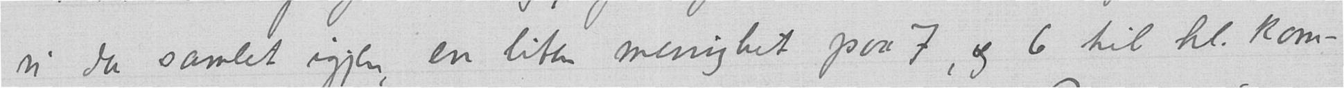vi da samlet igjen, en liten menighet paa 7, og 6 til hl. kom-
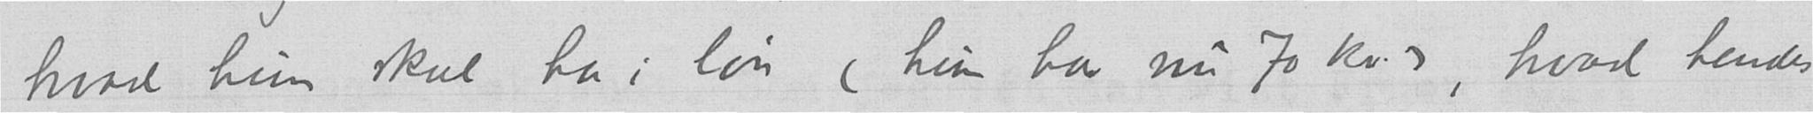hvad hun skal ha i løn (hun har nu 70 kr.), hvad hendes
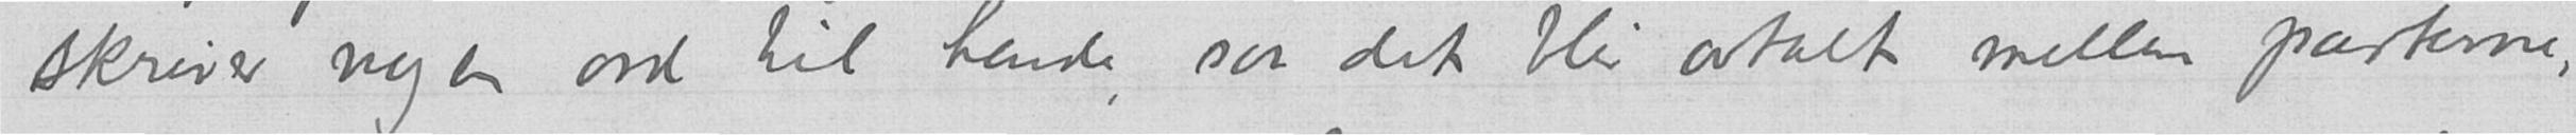skriver nogen ord til hende, saa det blir avtalt mellem parterne,
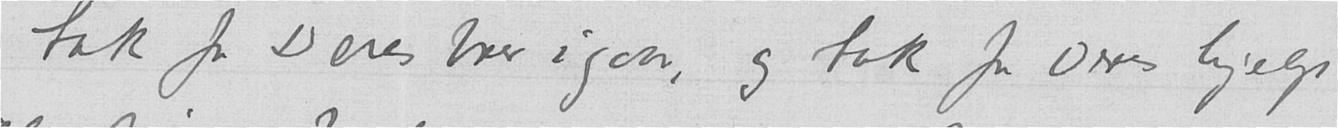tak for Deres brev igaar, og tak for Deres hjelp
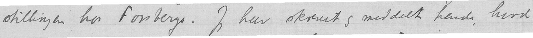stillingen hos Forsbergs. Jeg har skrevet og meddelt hende, hvad
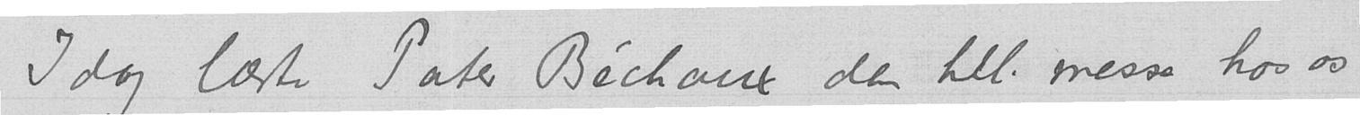I dag læste Pater Bechaux den hll. messe hos os
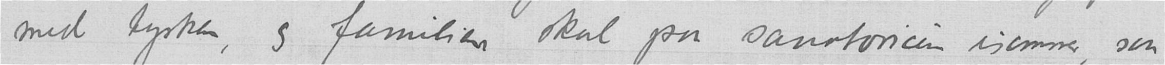med tysken, og familien skal paa sanatorium isommer, saa
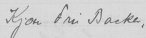Kjære Fru Backer,
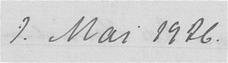1. Mai 1926.
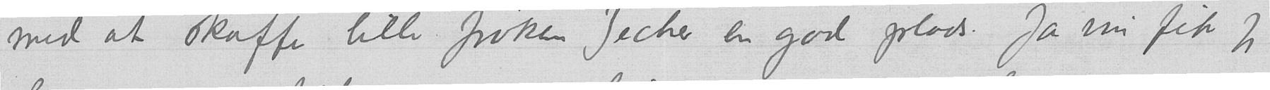med at skaffe lille frøken Zecher en god plads. Ja nu fik jeg
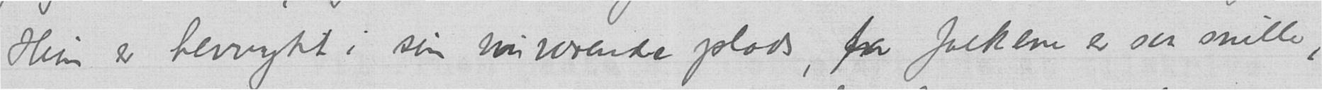Hun er henrykt i sin nuværende plads, for folkene er saa snille,
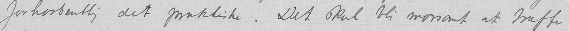forhaabentlig det praktiske. Det skal bli morsomt at træffe
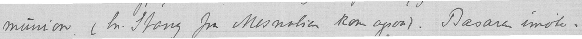munion (hr. Stang fra Mesnalien kom ogsaa). Basaren imøte-
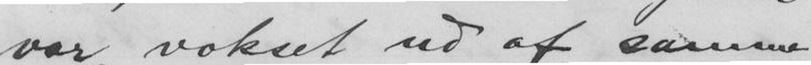var vokset ud af samme
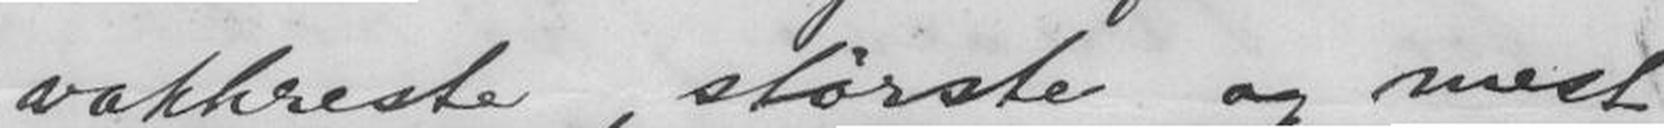vakkreste, største og mest
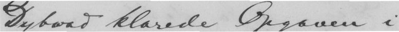Dybwad klarede Opgaven i
